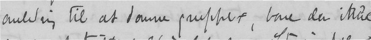anledning til at danne grupper, bare der ikke
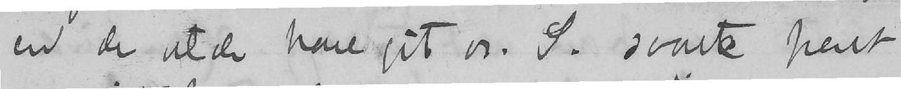end de vilde have git os. S. svarte pent
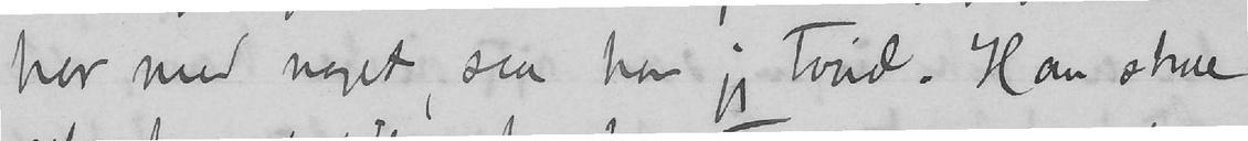har med noget, saa har jeg tvivl. Han skal
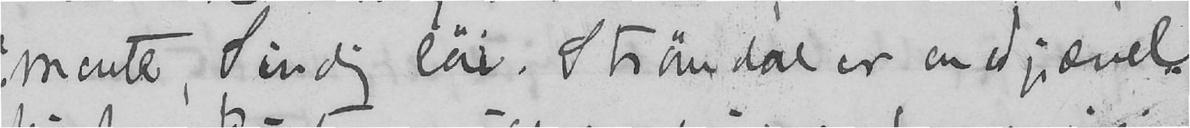mente Sinding løi. Strømdal er en djævel.
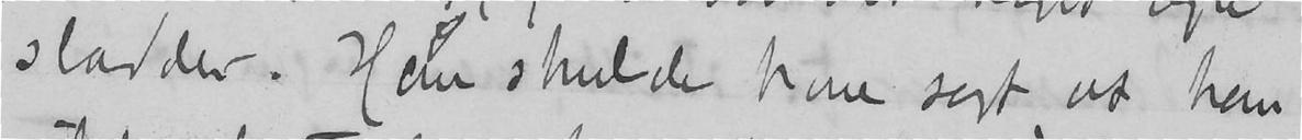sladder. Han skulde have sagt, at han
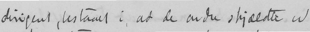dirigent, bestaaet i, at de andre skjældte ud
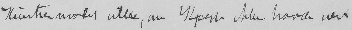Kunstnermødet vilde, om Krogh ikke havde vært
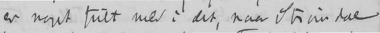er noget fult med i det, naar Strømdal
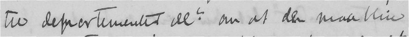til departementet om at der maa blive
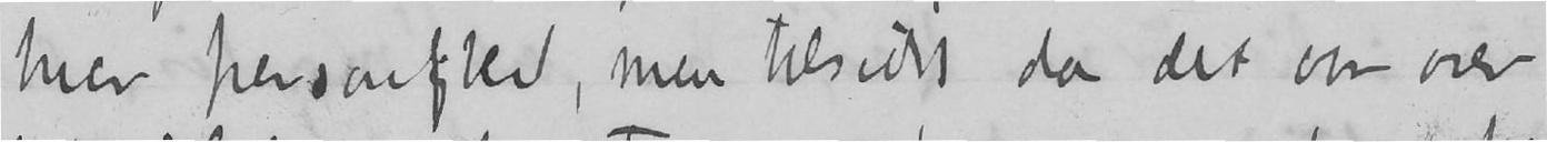hver personheds, men tilsidst da det var over
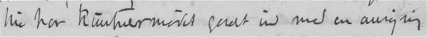Nu har Kunstnermødet gaat ind med en ansøgning
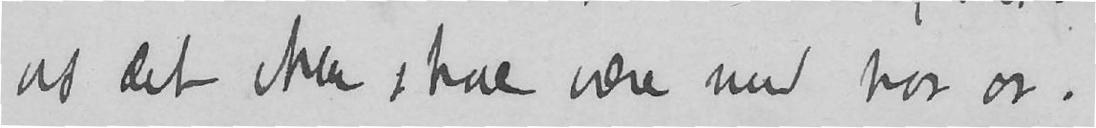at det ikke skal være med hos os.
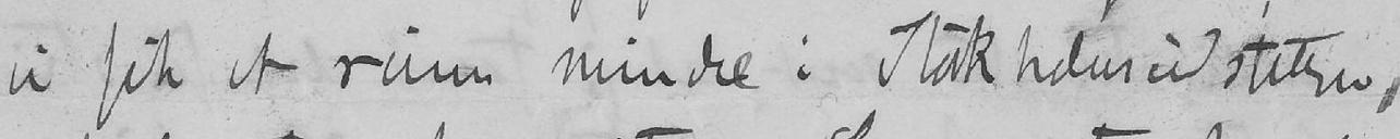vi fik at rum mindre i Stockholmudstillingen,
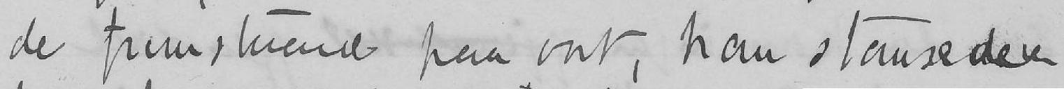de fremskuende paa ondt, han stansede
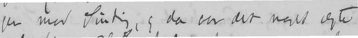ger mod Sinding, og da var det noget ægte
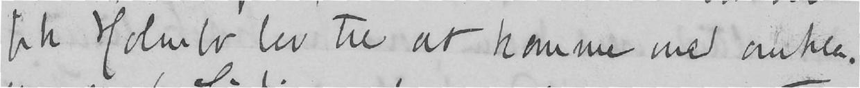fik Holmbo lov til at komme med ankla-
